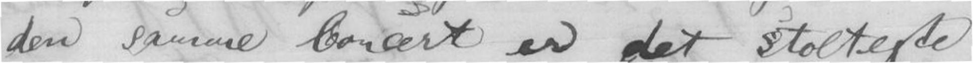den samme Concert er det stolteste
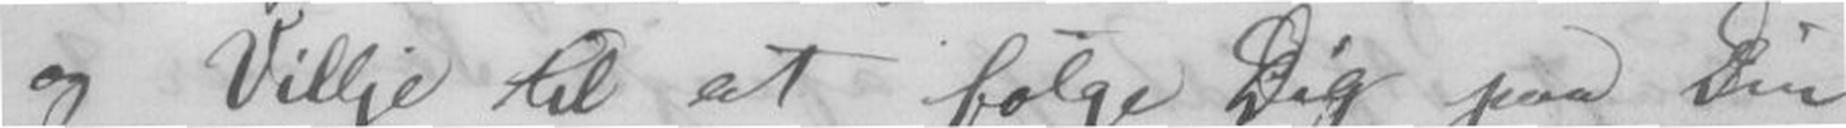og Villje til at følge Dig paa Din
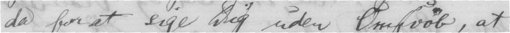da for at sige Dig uden Omsvøb, at
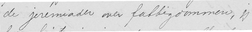de jeremiader over fattigdommen, jeg
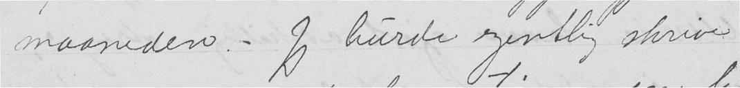maaneden. - Jeg burde egentlig skrive
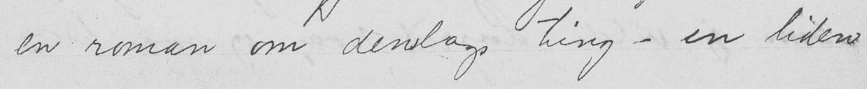en roman om denslags ting - en liden
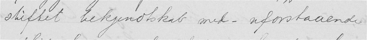stiftet bekjendtskab med - uforstaaende
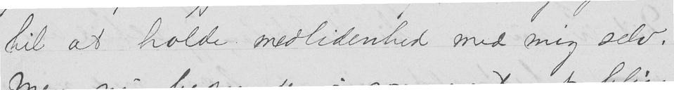til at holde medlidenhed med mig selv.
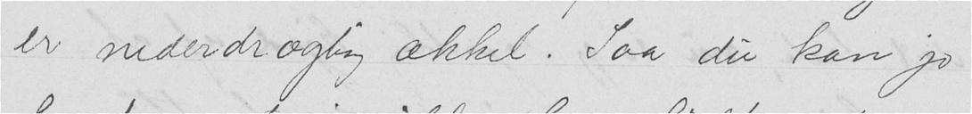er nederdræktig ækkel. Saa du kan jo
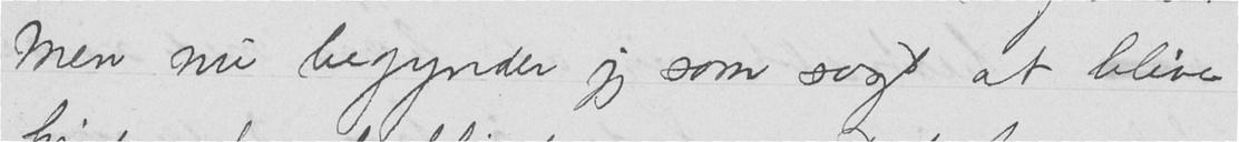Men nu begynner jeg som sagt at blive
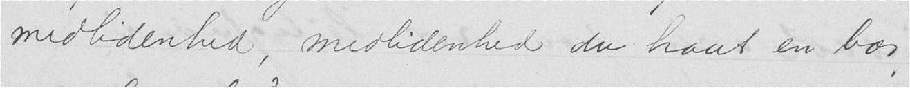medlidenhed, medlidenhed du haut en bas,
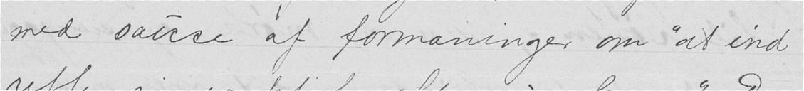med sauce af formaninger om "at ind-
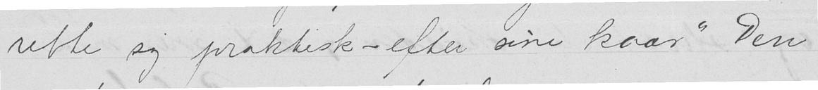rette sig praktisk - efter sine kaar" Den
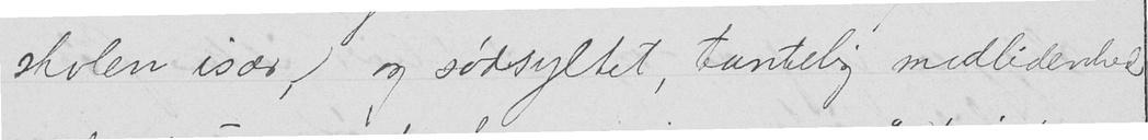skolen især), og sødsyltet, tantelig medlidenhed
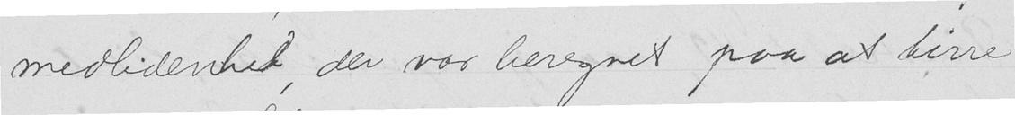medlidenhed, der var beregnet paa at tirre
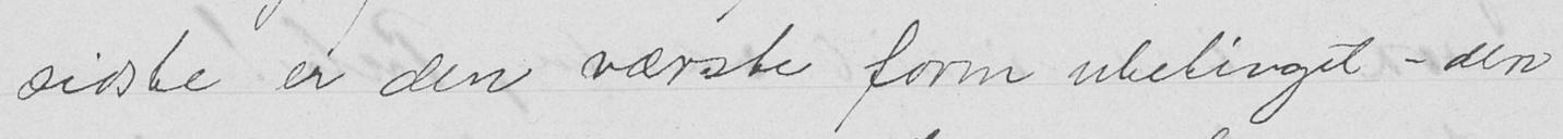sidste er den værste form ubetinget - den
